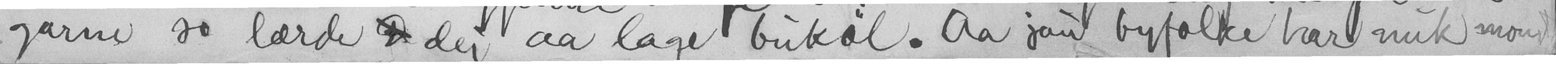garne so lærde  dei aa lage bukøl. Aa jau byfolke har nuk mom
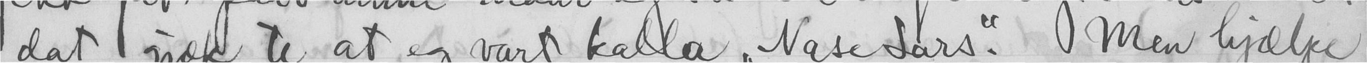dat gjæk te at eg vart kalla "NaseLars". Men hjælpe
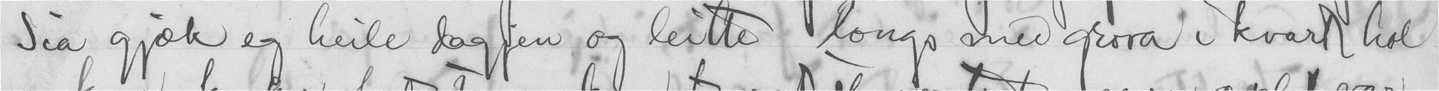Saa gjæk og heile dagjen og leitte longs med grova i kvart hol
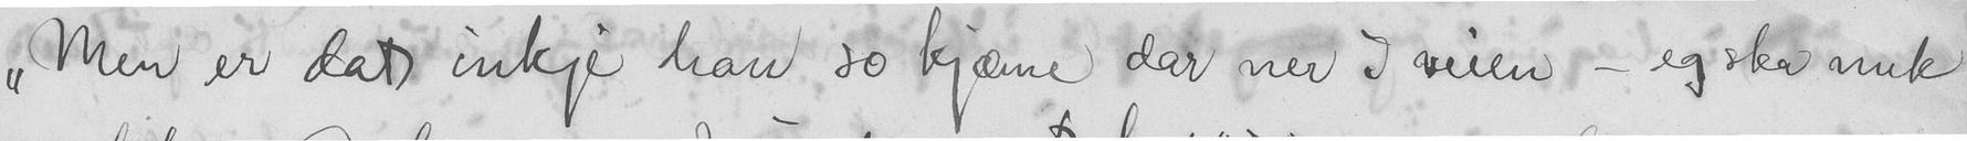"Men er dat inkje han jo kjæme dar ner i veien, - eg ska nuk
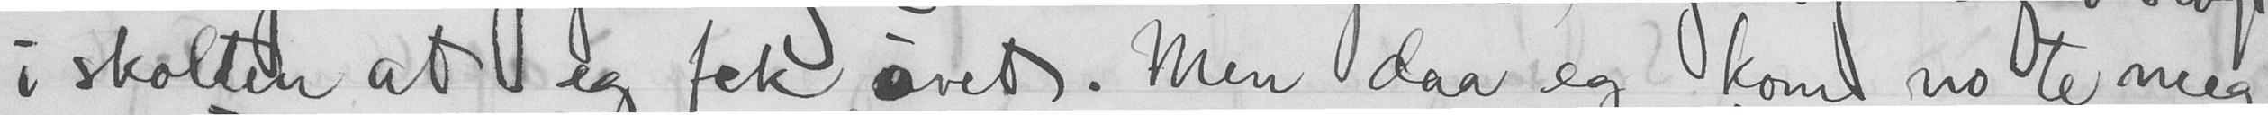i skolten at eg fek uvet. Men daa og kom no te meg
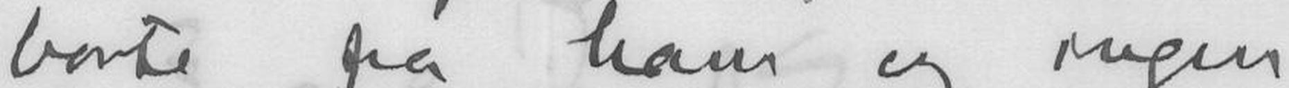borte fra ham og ingen
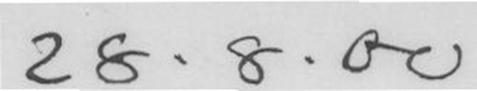28.8.00
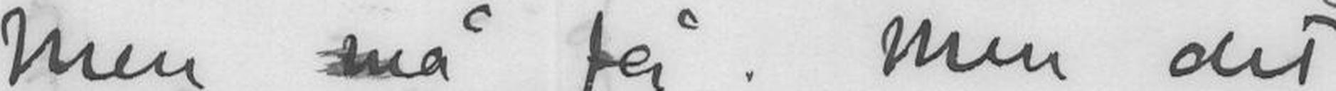Men må få. Men det
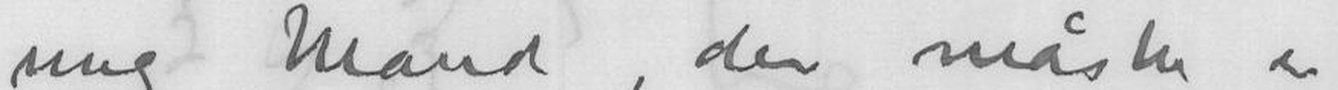ung Mand, der måske er
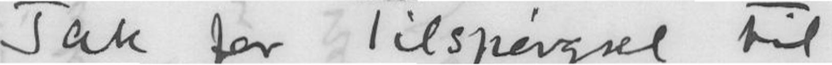Tak for Tilspørgsel til
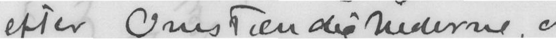efter Omstendighederne, og
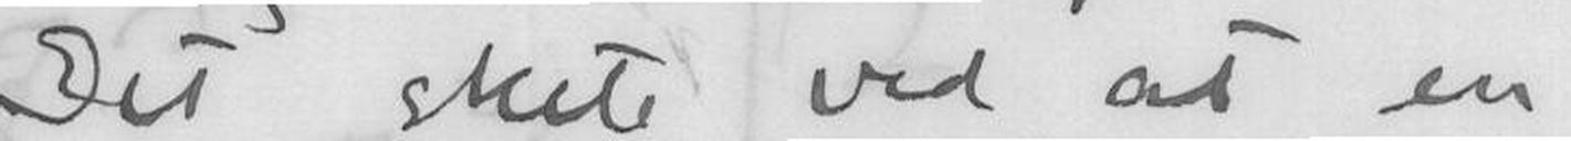Det skete ved at en
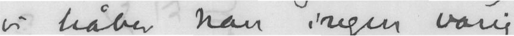vi håber han ingen varig
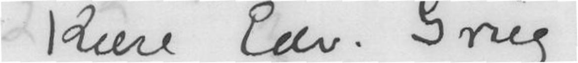Kære Edv. Grieg!
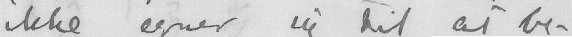ikke egner sig til at be-
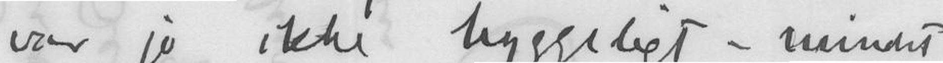var jo ikke hyggeligt - mindst
